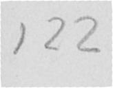152
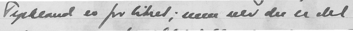Tyskland er forbitret; men selv der er det
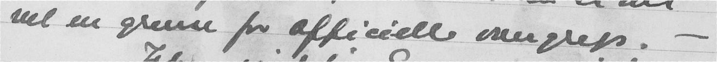vel en grense for officielle overgrep. -
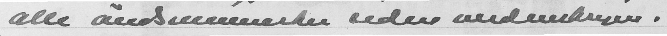alle åndsmennesker siden verdenskrigen.
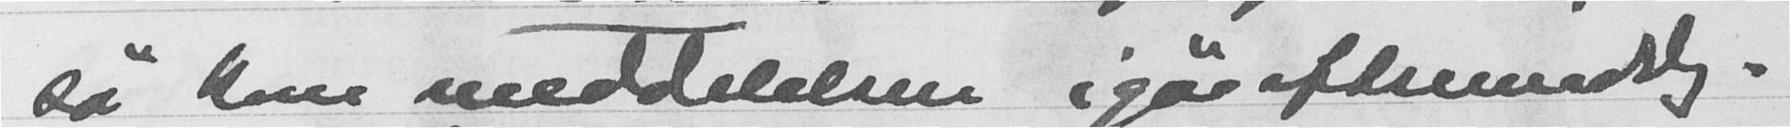så kom meddelelsen igår eftermiddag.
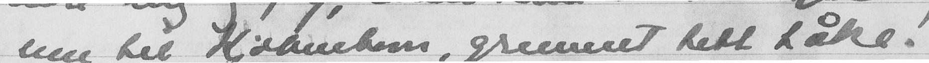enn til Kjøbenhavn, grunnet tett tåke!
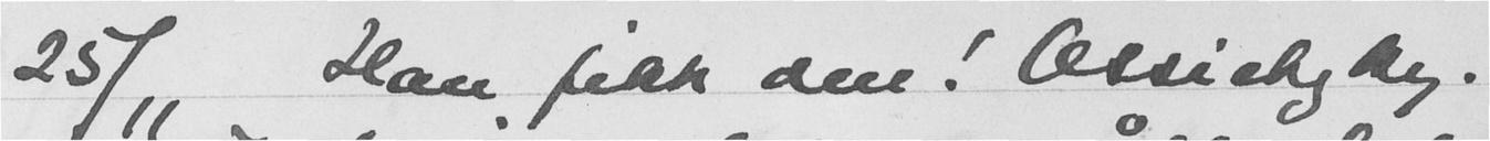25/11 Han fikk den. Ossietzky.
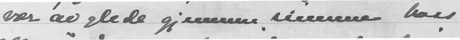vær av glede gjennem sinnene hoss
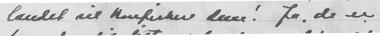landet vil konfiskere dem. Ja, de er
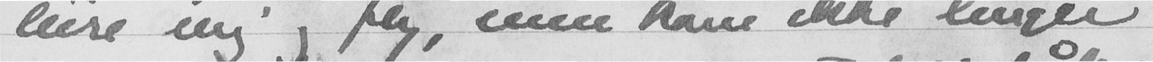lure mig og fly, men kom ikke lenger
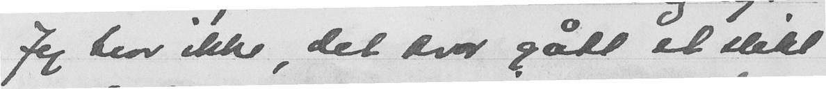Jeg tror ikke, det har gått et slikt
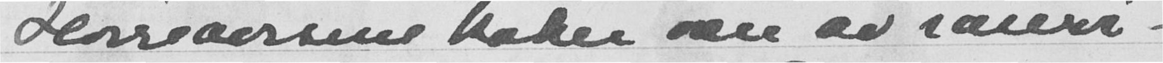Høireavisene later være av raseri
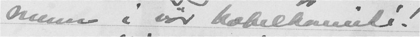menna i vår nokelkomité!
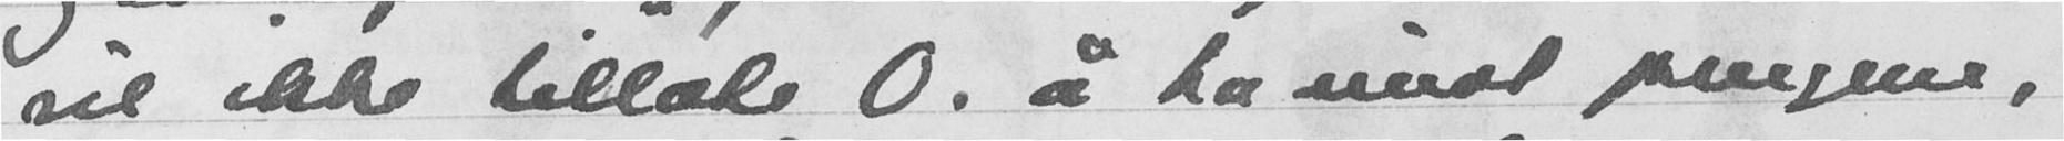vil ikke tillate O. å ta imot pengene,
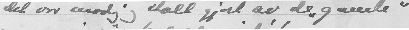Det var modig og stolt gjort av de "gamle"
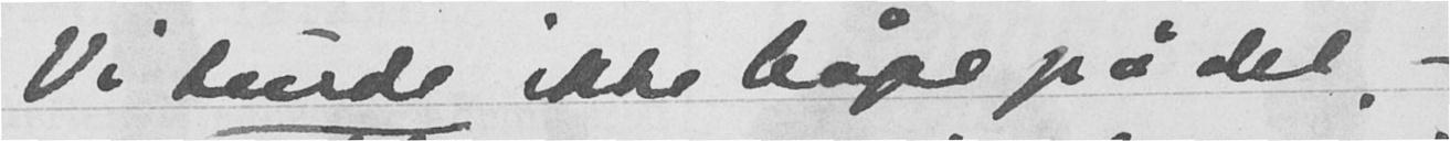Vi turde ikke håpe på det, -
